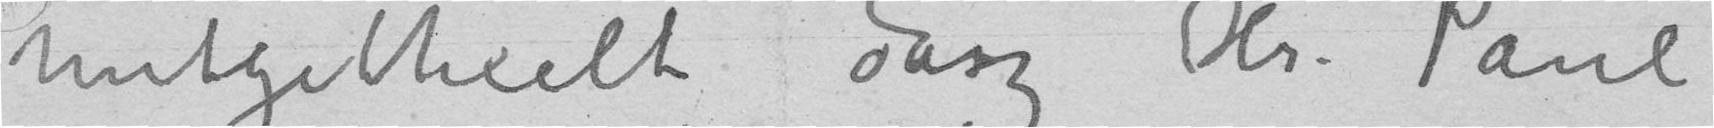mitgetheilt dasz Hr. Paul
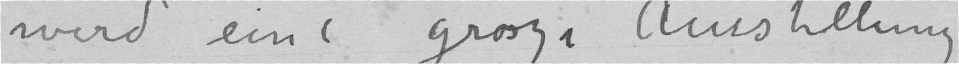wird eine grosze Ausstellung
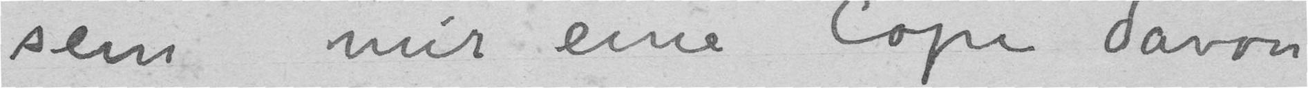sein mir eine Copi davon
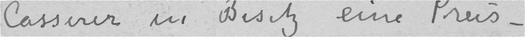Cassirer in Besitz eine Preis-
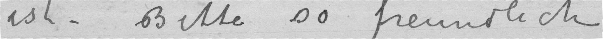ist – Bitte so freundlich
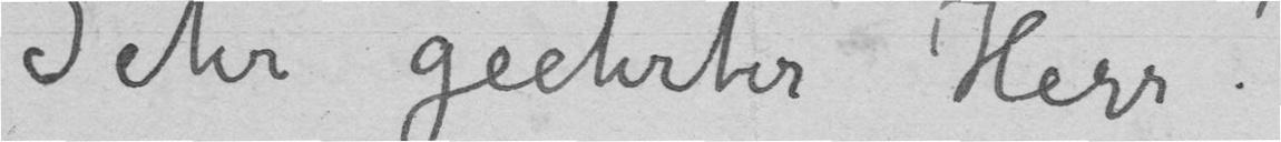Sehr geehrter Herr!
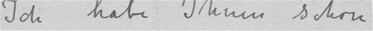Ich habe Ihnen schon
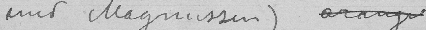und Magnussen) arangi
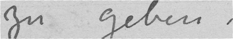zu geben.
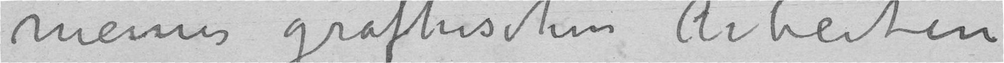meiner grafhischen Arbeiten
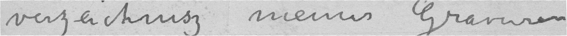verzeichnisz meiner Gravuren
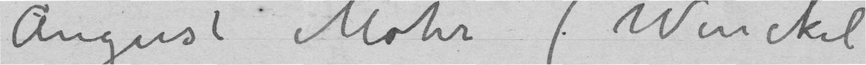August Mohr (Winckel
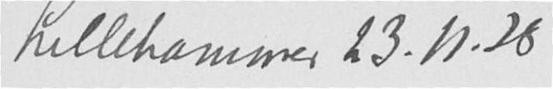Lillehammer 23.11.28
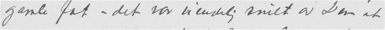gamle fat – det var uendelig snilt av Dem at
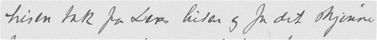tusen tak for Deres hilsen og for det skjønne
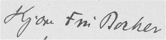Kjære Fru Backer
